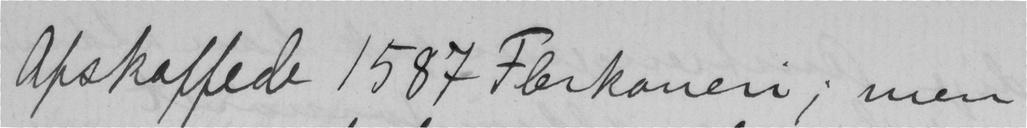Afskaffede 1587 Flerkoneri; men
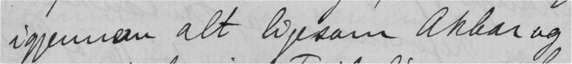igjennem alt ligesom Akbar og
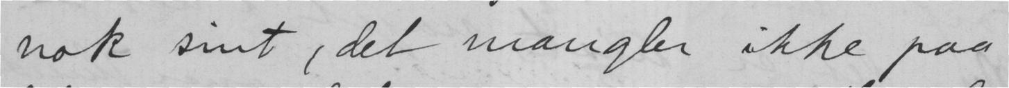nok sint, det mangler ikke paa
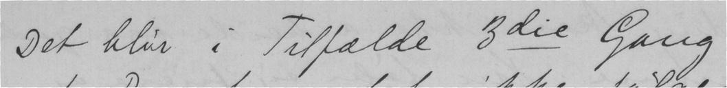Det blir i Tilfælde 3die Gang
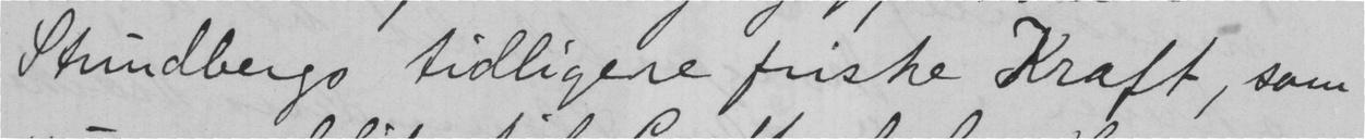Strindbergs tidligere friske Kraft, som
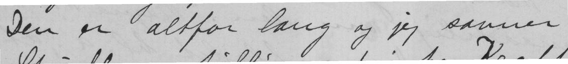Den er altfor lang og jeg savner
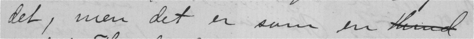det, men det er som en
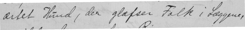ærtet Hund, der glæfser Folk i Læggene,
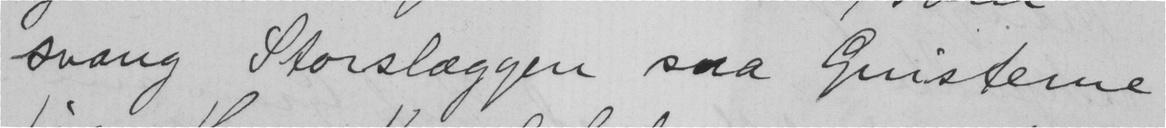svang Storslæggen saa Gnisterne
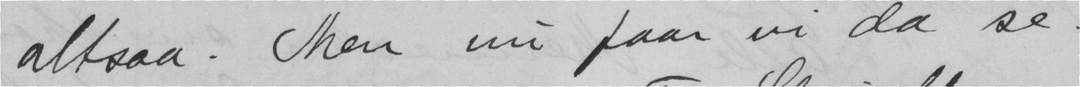altsaa. Men nu faar vi da se.
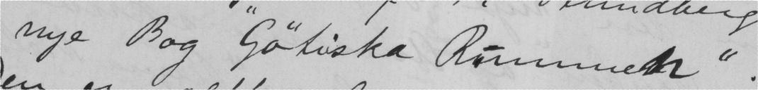nye Bog "Gøtiska Rummen".
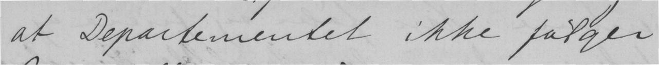at Departementet ikke følger
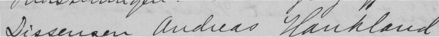Dissensen Andreas Haukland
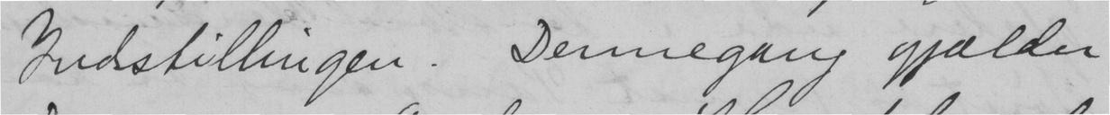Indstillingen. Dennegang gjælder
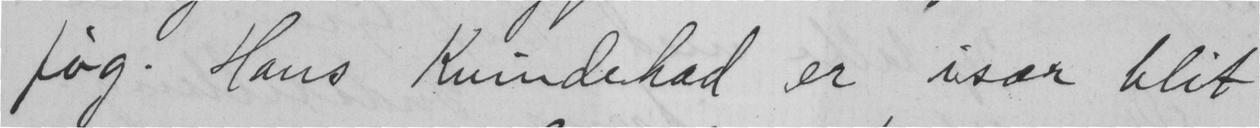føg. Hans Kvindehad er især blit
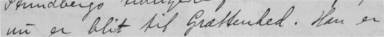nu er blit til Grættenhed. Han er
